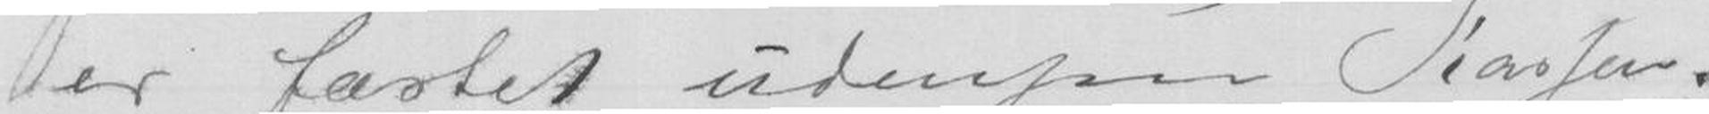er fæstet udenpaa Kassen.
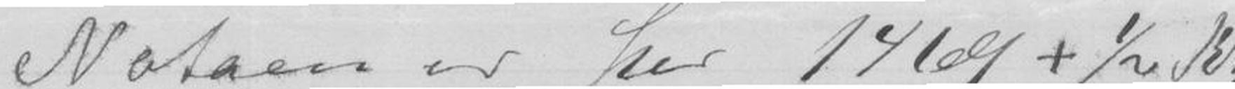Notaen er stor 1469 + 1/2 Kr
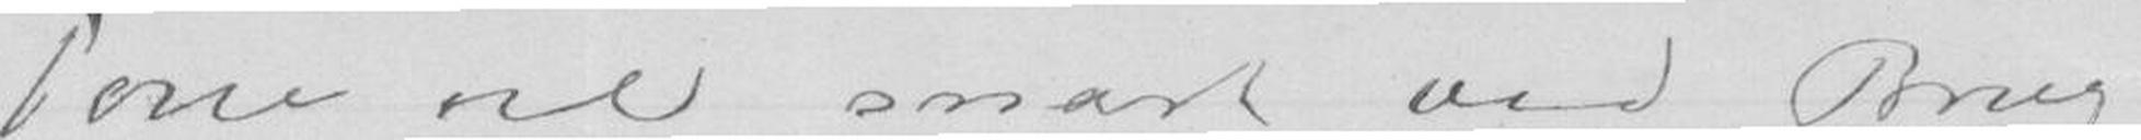Tone vil snart ved Brug
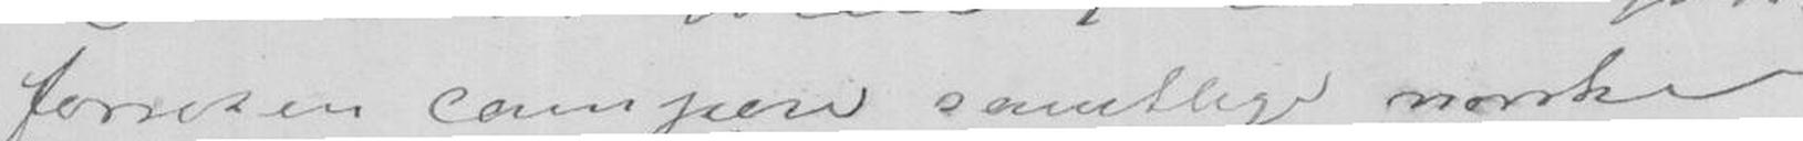foresten campere samtlige norske
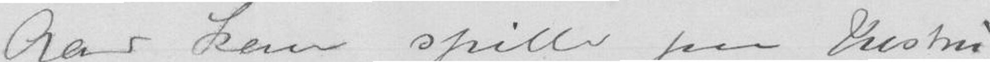Aar kan spille paa Instru-
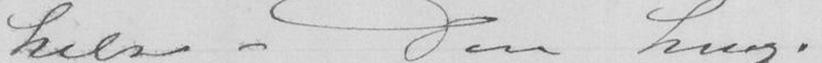hilse. Din heng.
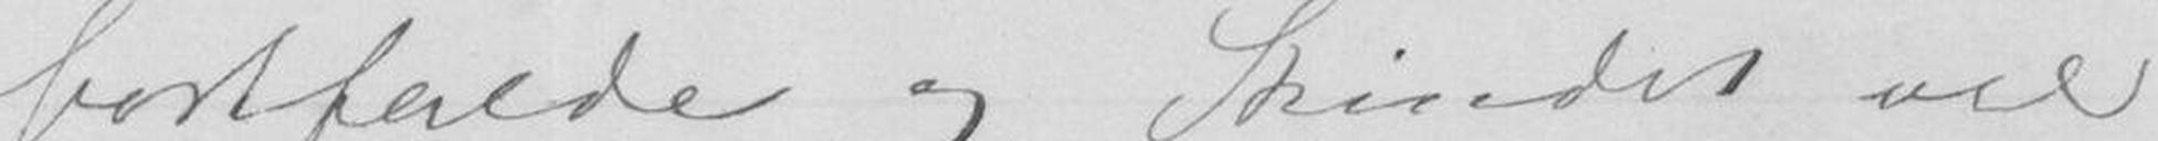bortfalde og Skindet vil
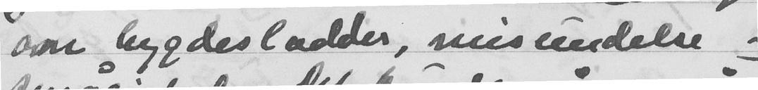om bygdesladder, misundelse og
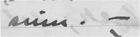sinn. -
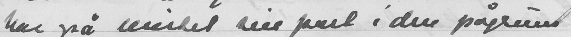har også mistet sin part i den pågrunn
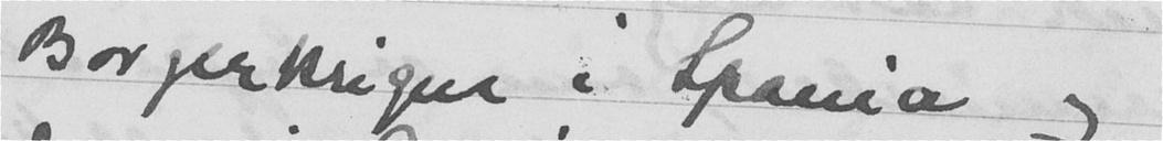Borgerkrigen i Spania og
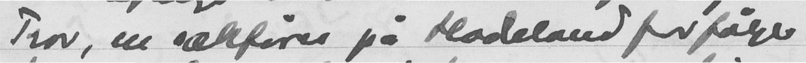Tror, en sakfører på Hadeland forfølger
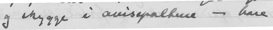og skygge i avisspaltene - bare
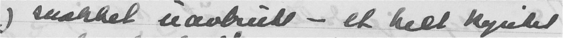og snakket uavbrutt - et helt kyntet
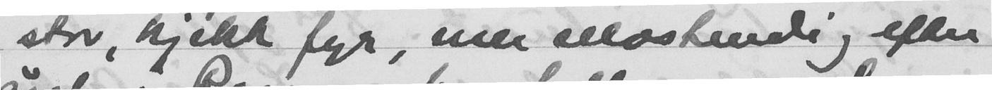stor, kjekk fyr, ver selvstendig efter
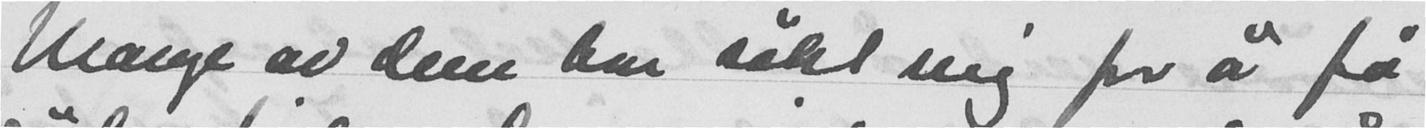Mange av dem har søkt mig for a få
